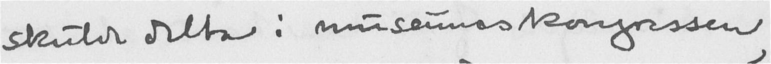skulde delta i museumskongressen,
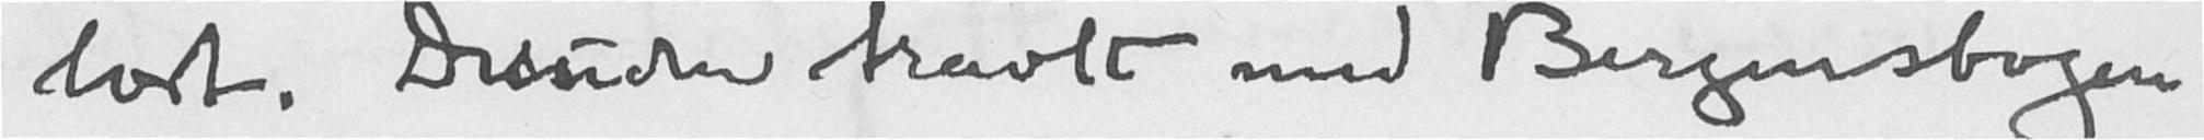lort. Desuden travlt med Bergensbogen.
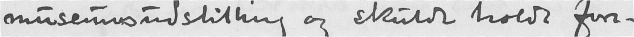museumudstilling og skulde holde fore-
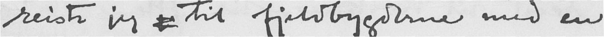reiste jeg til fjeldbygderne med en
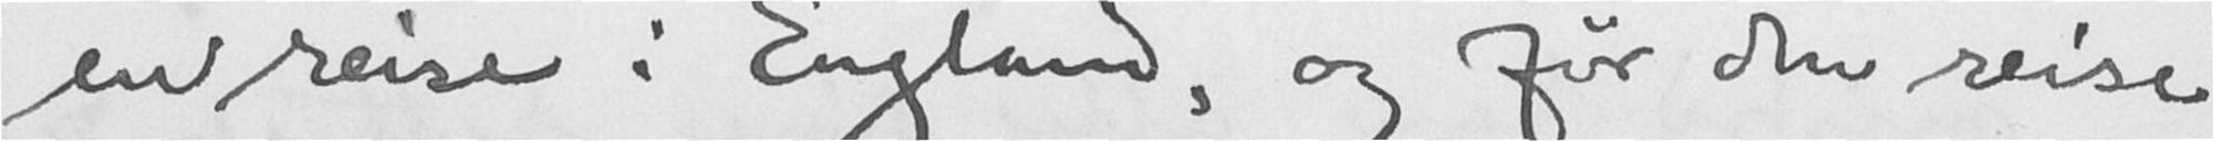en reise i England, og før den reise
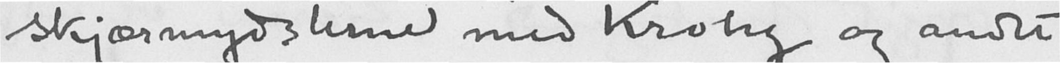skjærmydslerne med Krohg og andet
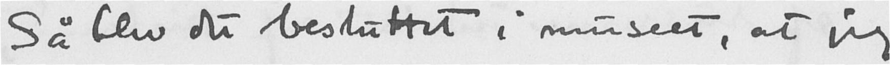Så blev det besluttet i museet, at jeg
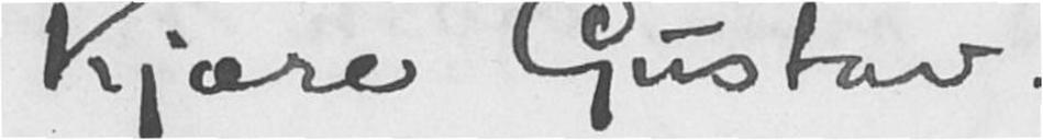Kjære Gustav.
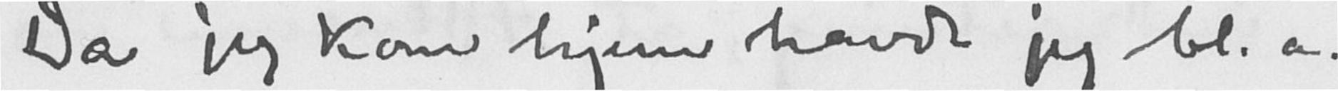Da jeg kom hjem hvade jeg bl.a.
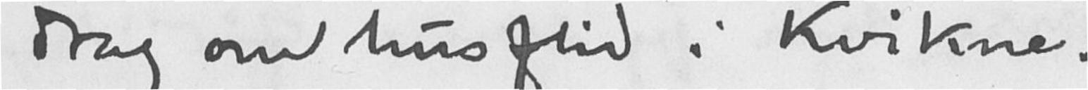drag om husflid i Kvikne.
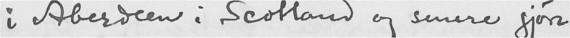i Aberdeen i Scotland og senere gjøre
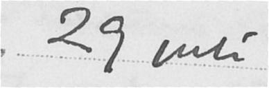29 juli
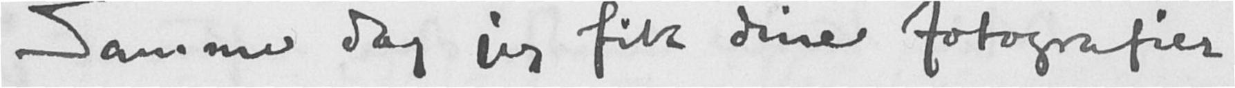Samme dag jeg fik dine fotografier
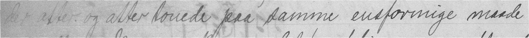der after og after tonede paa samme ensformige maade
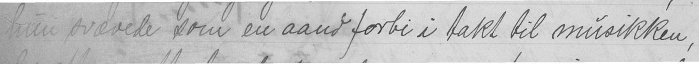hun svævede som en aand forbi i takt til musikken,
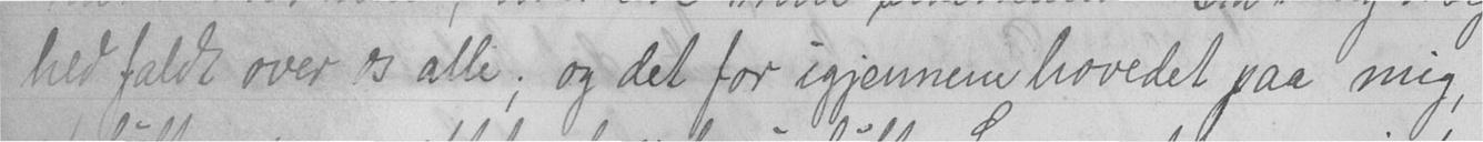hed faldt over os alle; og det for igjennem hovedet paa mig,
